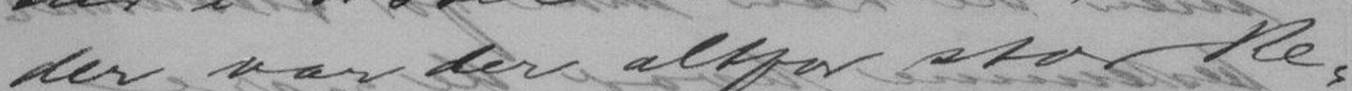der var der altfor stor Re-
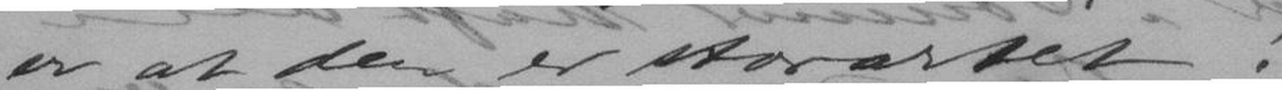er at den er storartet!
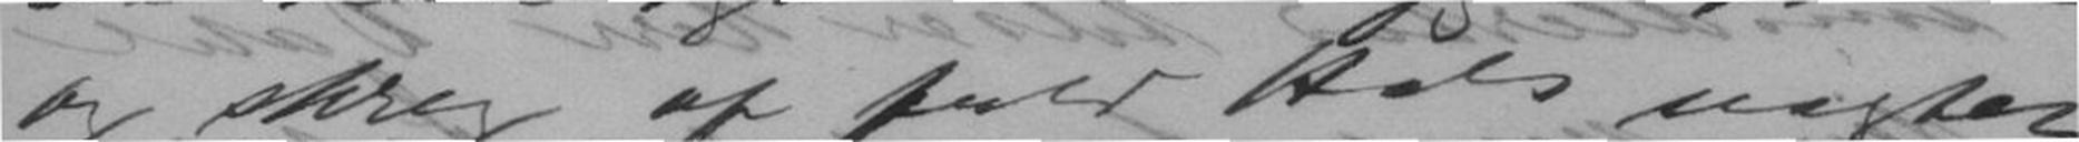og skreg af fuld Hals nægtet
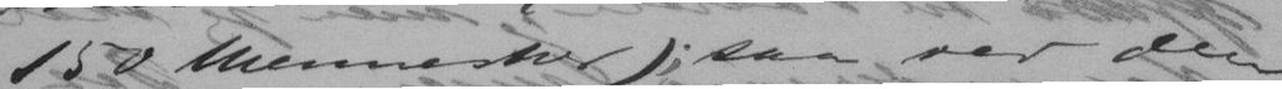150 Mennesker); saa ved den
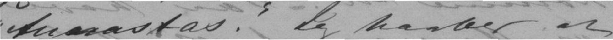"Annastas". Jeg haaber at
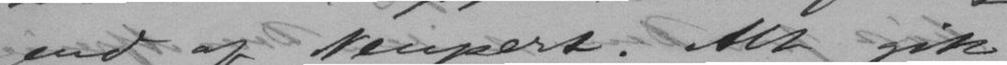end af Neupert. Alt gik
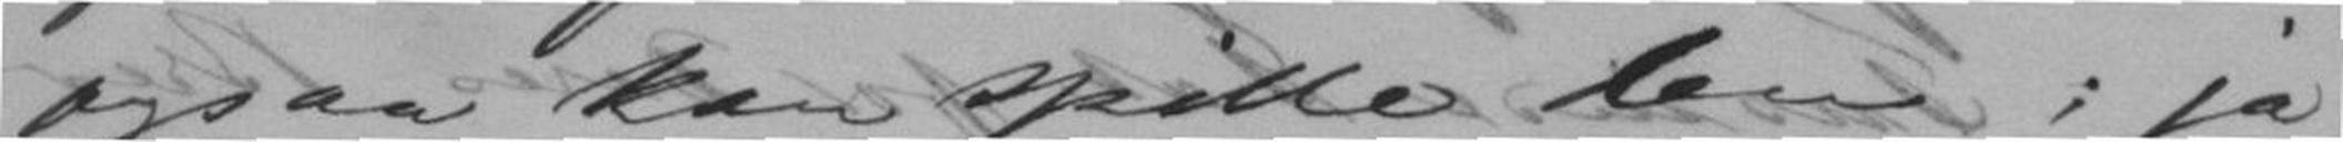ogsaa kan spille den; ja
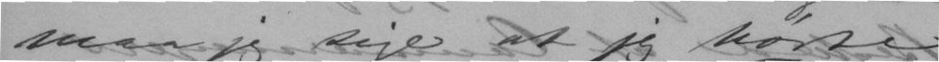maa jeg sige at jeg hørte
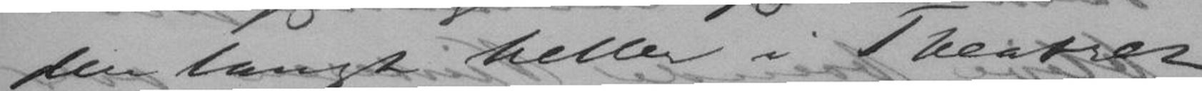den langt heller i Theatret
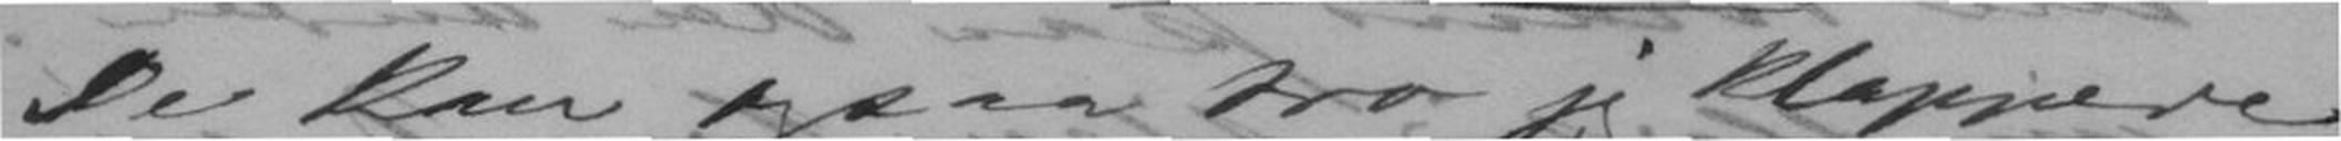De kan ogsaa tro jeg klappede
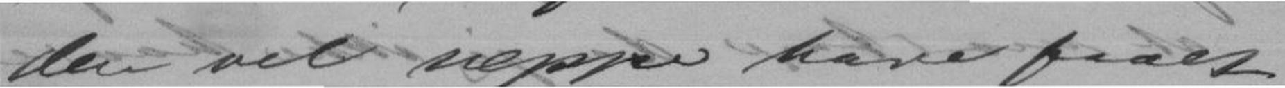den vel neppe have faaet
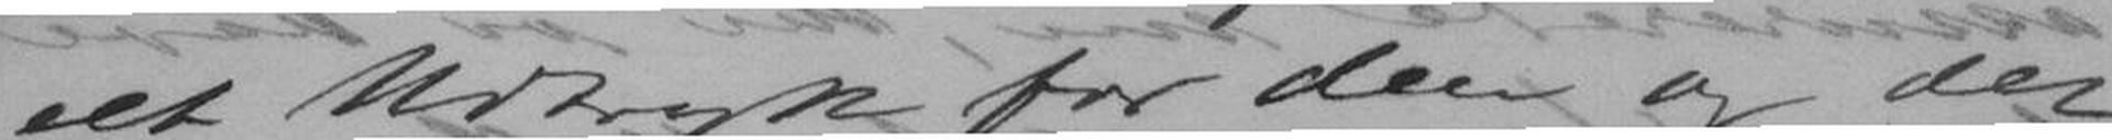alt Udtryk for den og det
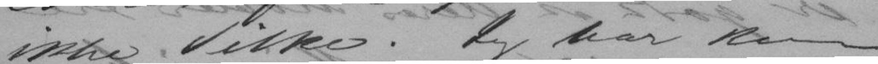ikke Silke. Jeg har kun
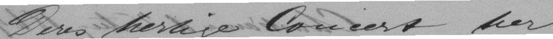Deres herlige Concert her
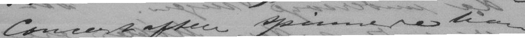Consertaften spinnede lian
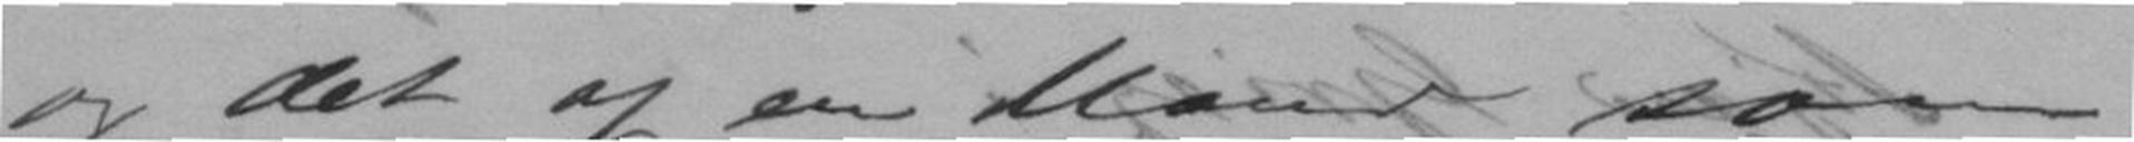og det af en Mand som
